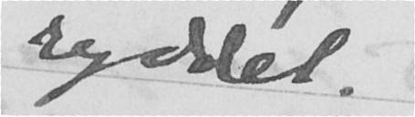ryddet.
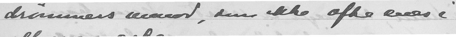drømmers mand, som ikke ofte sees i
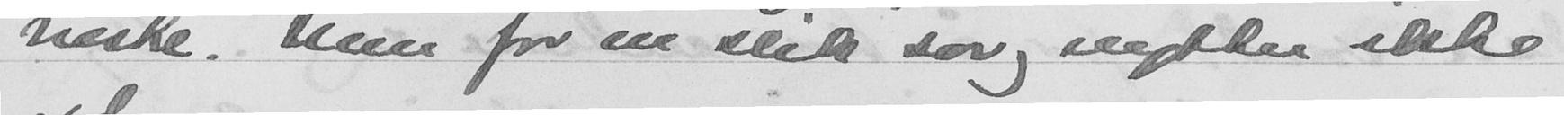neske. Men for en slik sorg nytter ikke
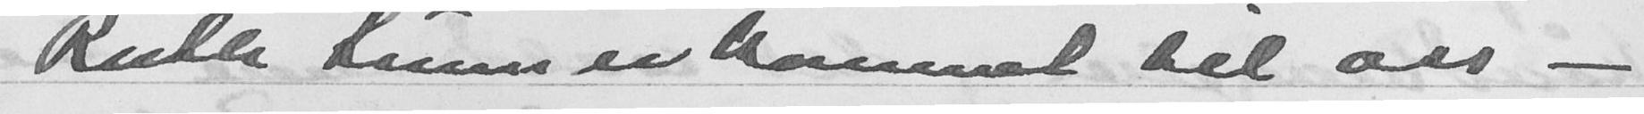Ruth Leum kommet til oss -
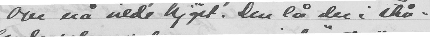Ove nå vilde kjøpt. Den lå der i strå-
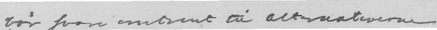bør svare omtrent til alternativerne
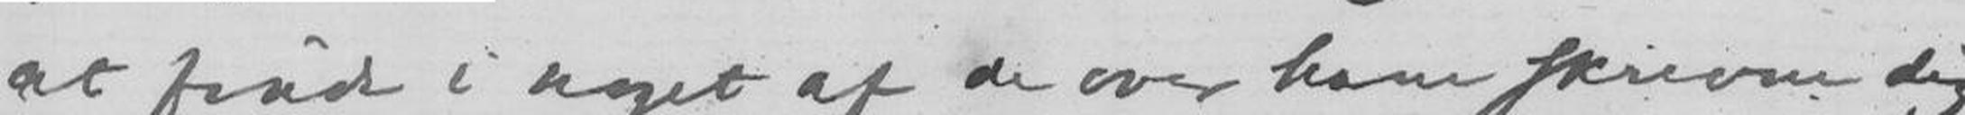at finde i noget af de over ham skrevne dig-
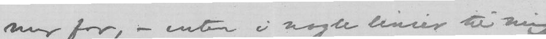mer for, - enten i nogle linier til mig
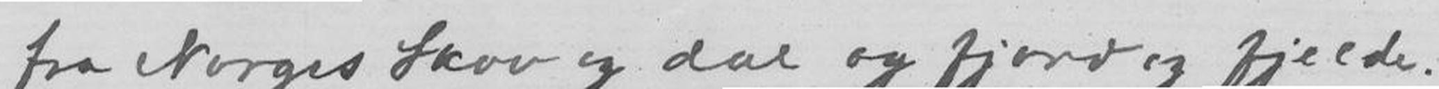fra Norges Skov og dal og fjord og fjelde.
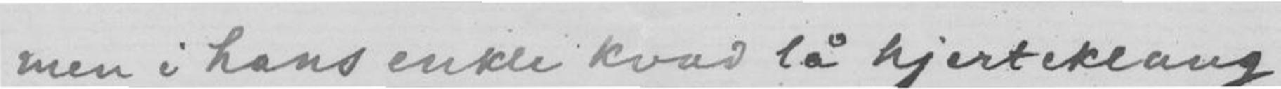men i hans enkle kvad lå hjerteklang
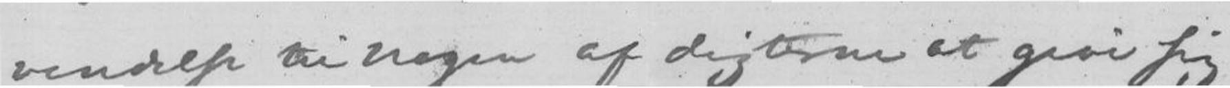vendelse til nogen af digterne at give sig
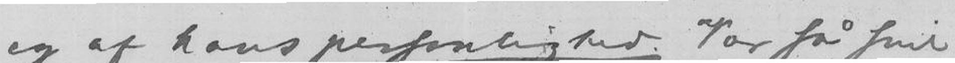og af hans personlighed. Vær så snil
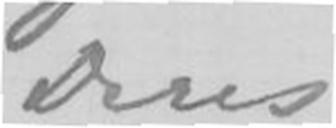Deres
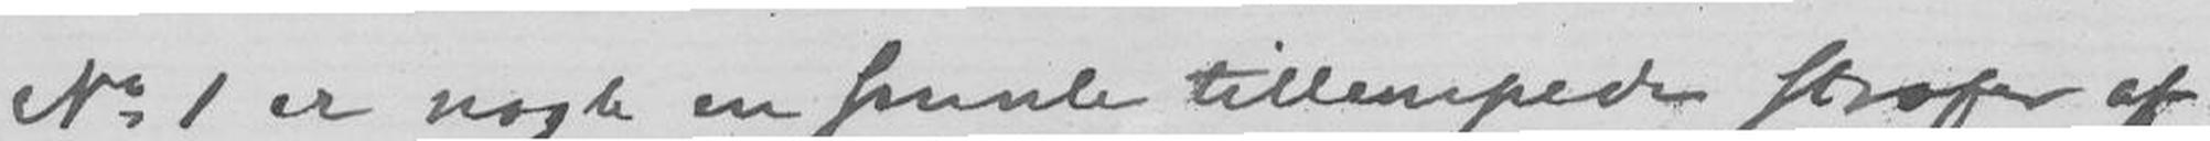Nr 1 er nogle en smule tillempede strofer af
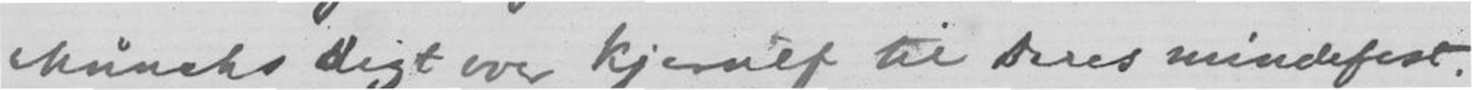Munchs Digt over Kjerulf til Deres mindefest.
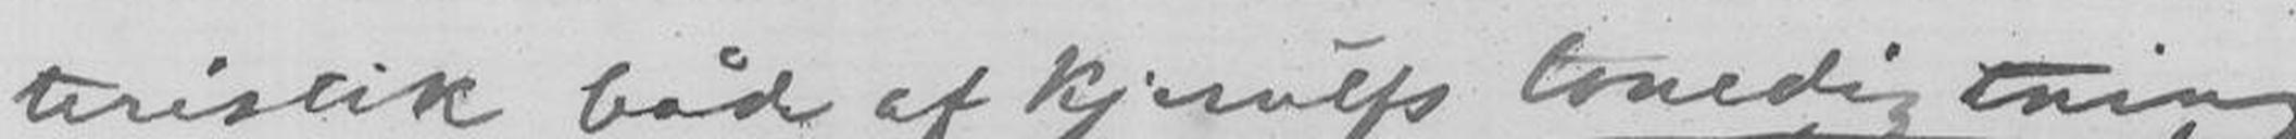teristik både af Kjerulfs tonedigtning
